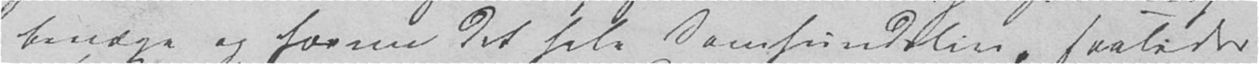bevæge og forme det hele Samfundsliv, saaledes
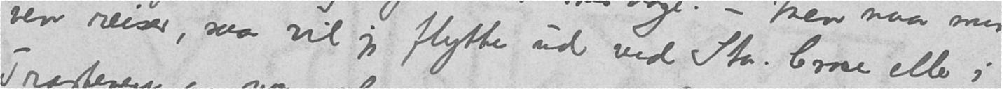ven reiser, saa vil jeg flytte ud ved Sta. Crose eller i
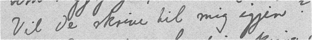Vil De skrive til mig igjen?
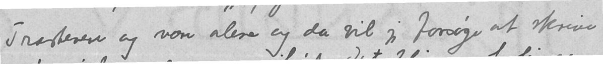Trastevere og være alene og da vil jeg forsøge at skrive
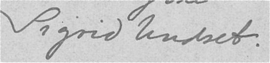Sigrid Undset.
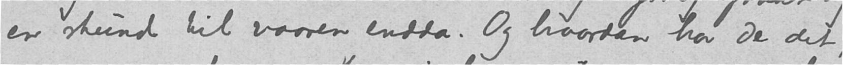en stund til vaaren endda. Og hvordan har De det,
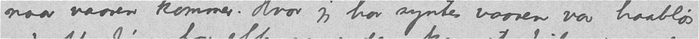naar vaaren kommer. Hvor jeg har syntes vaaren var haabløs
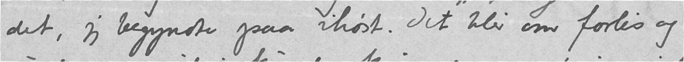det, jeg begyndte paa ihøst. Det blir om forlis og
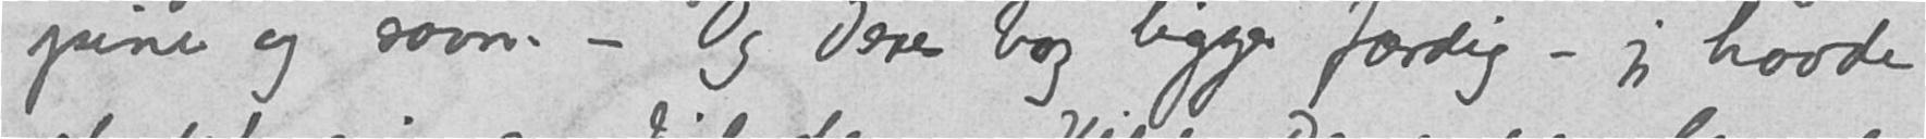pine og savn. - Og Deres bog ligger færdig - jeg havde
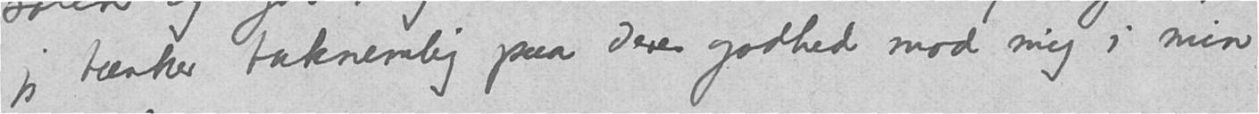jeg tænker taknemlig paa Deres godhed mod mig i min
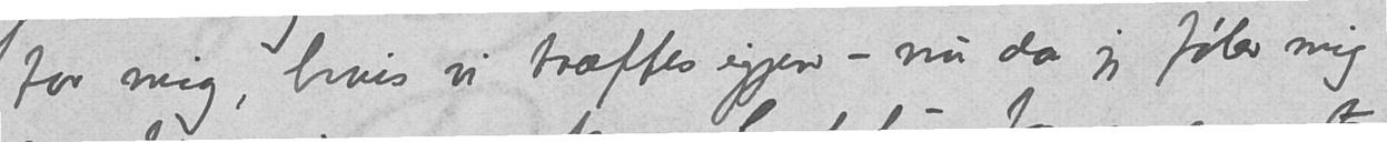for mig, hvis vi træffes igjen - nu da jeg føler mig
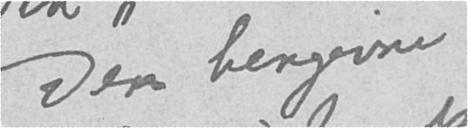Din hengivne
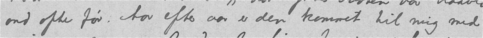ond ofte før: Aar efter aar er den kommet til mig med
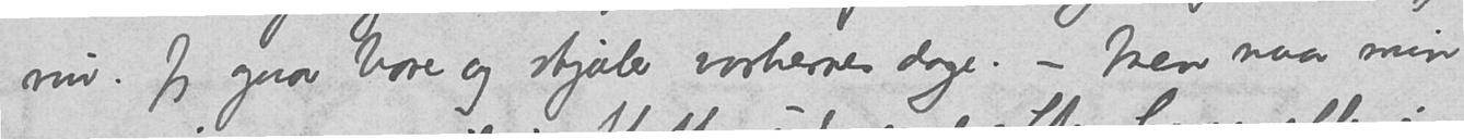nu. Jeg gaar bare og stjæler vorherres dage. - Men naar min
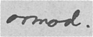armod.
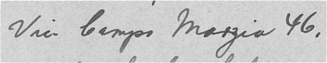Via Campo Marzia 46,
